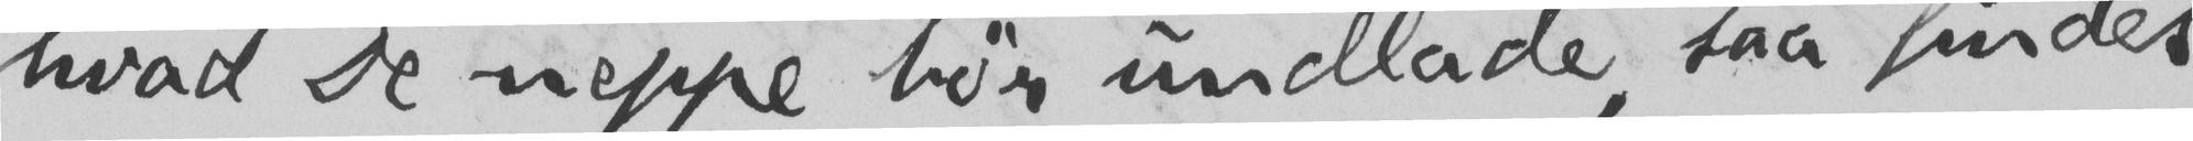hvad De neppe bør undlade, saa findes
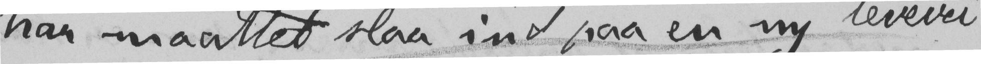har maattet slaa ind paa en ny levevei
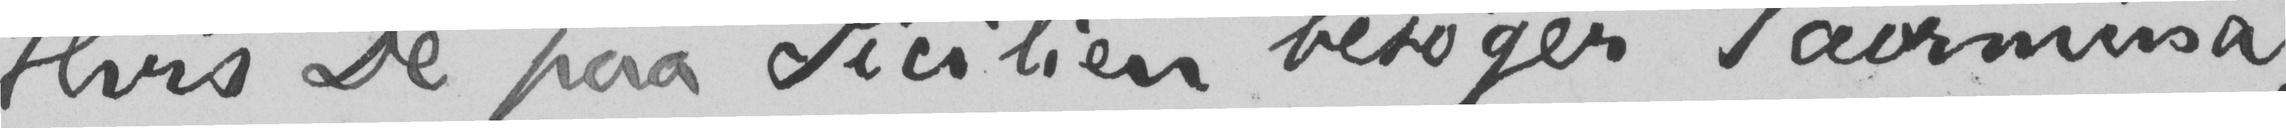Hvis De paa Sicilien bøsøger Taormina,
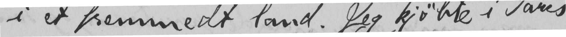i et fremmedt land. Jeg kjøbte i Paris
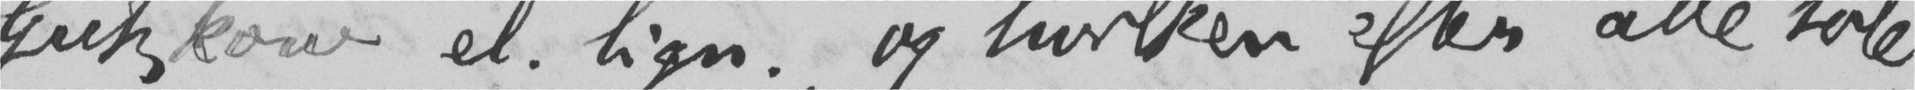Gutzkow el. lign., og hvilken efter alle sole-
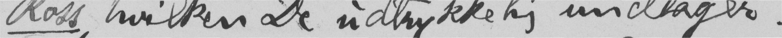Ross, hvilken De udtrykkelig undtager.
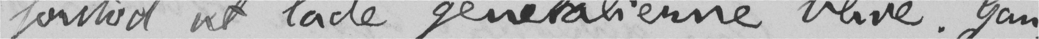forstod at lade genitalierne blive. Gan-
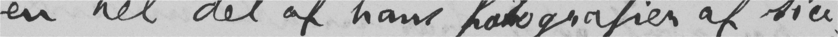en hel del af hans fotografier af sici-
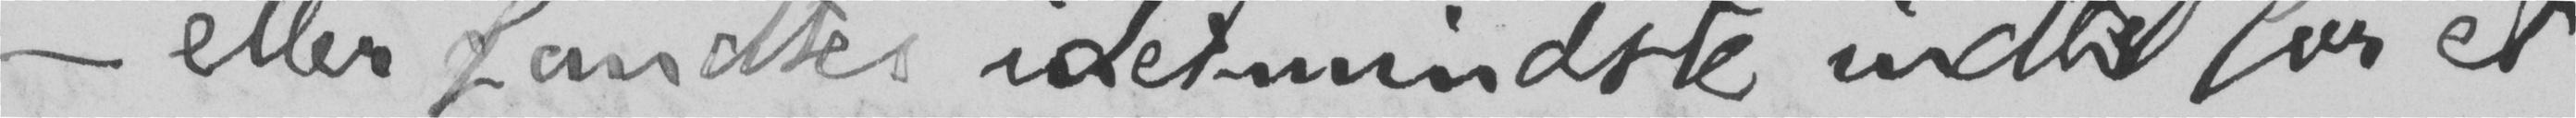– eller fandtes idetmindste intdil for et
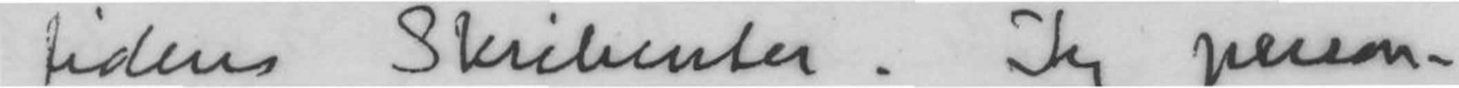tidens Skribenter. Jeg person-
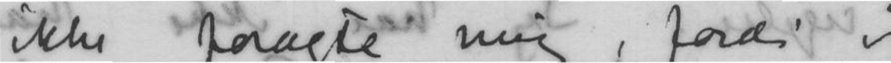ikke foragte mig, fordi jeg
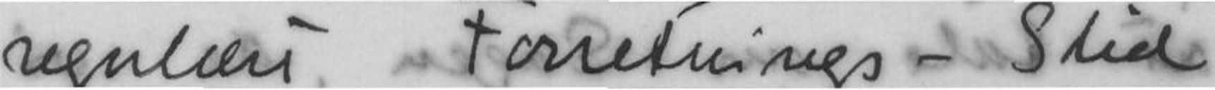regulært Forretnings - Slid,
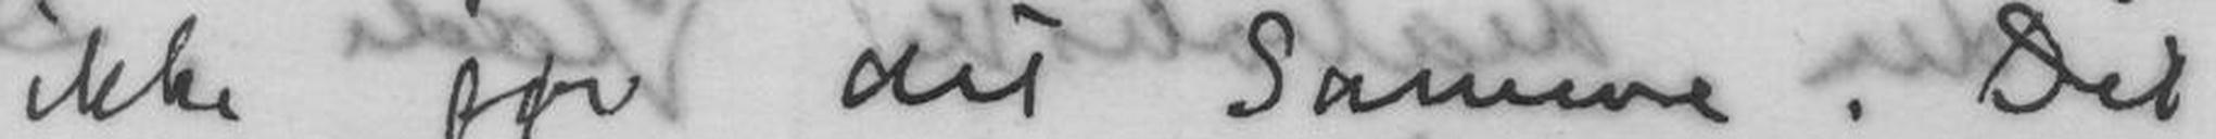ikke gør det Samme. Det
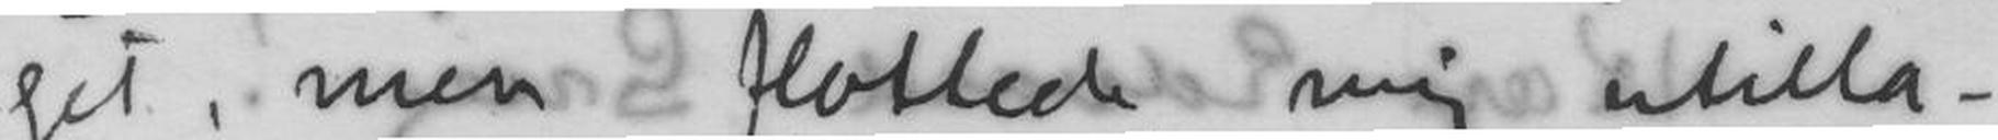get, men flottede mig utilla-
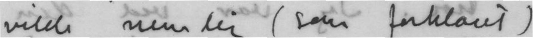vilde nemlig (som forklaret)
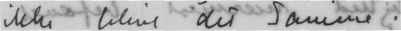ikke blive det samme.
